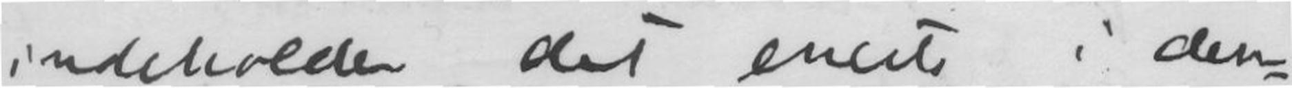indeholder det eneste i den-
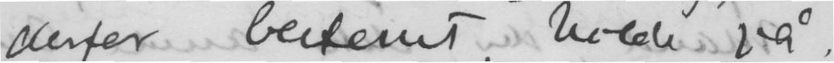derfor bestemt holde på
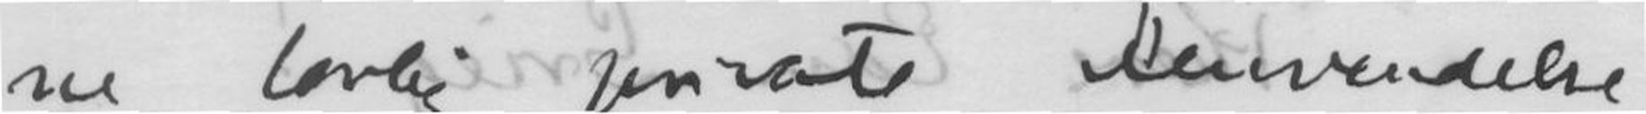ne lovlige private Henvendelse
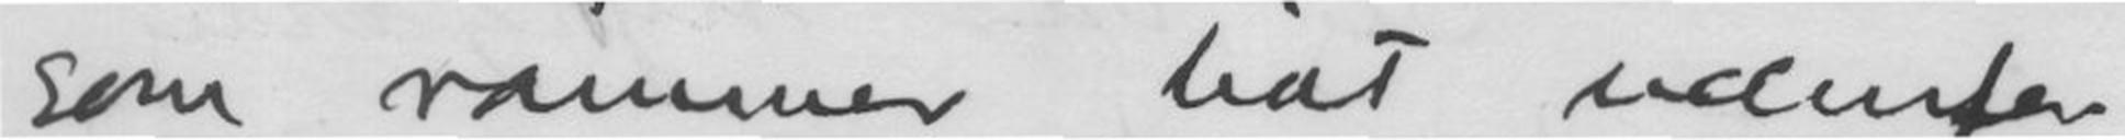som rammer lidt udenfor
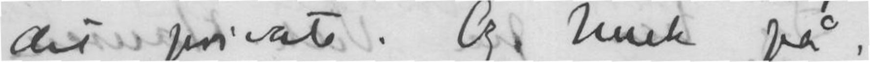det private. Og husk på
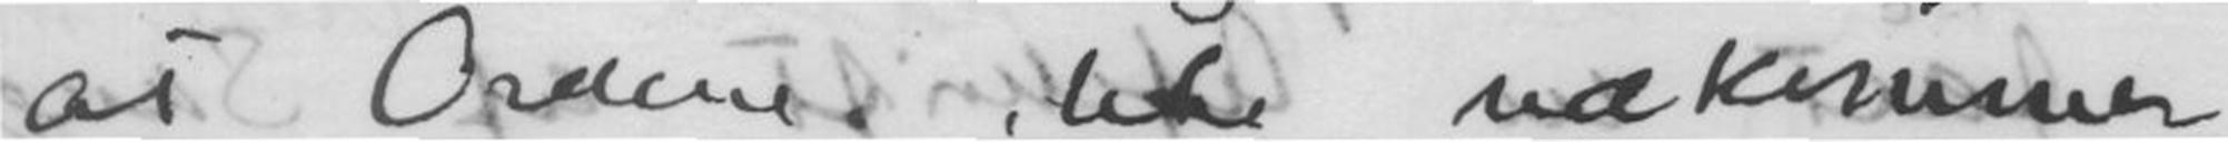at Ordene ikke udkommer
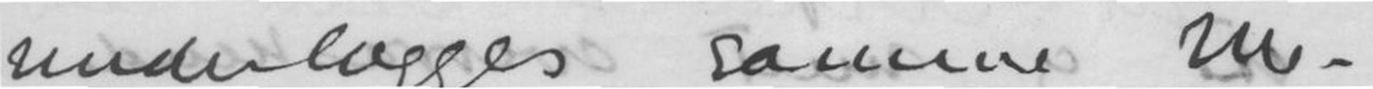underlægges samme Me-
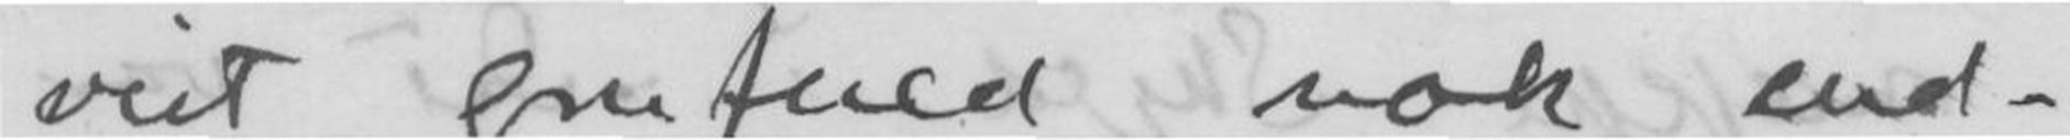vist grufuld nok end-
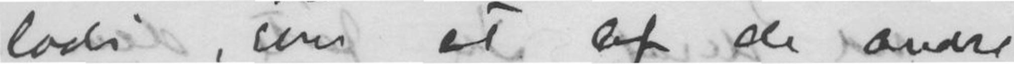lodi, som at af de andre
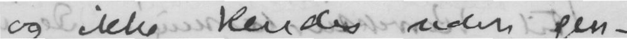og ikke kendes uden gen-
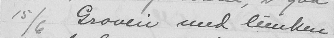15/6 Groveir med lunken
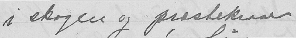i skogen og præstekraver
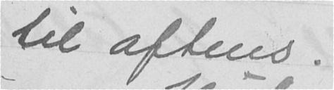til aftens.
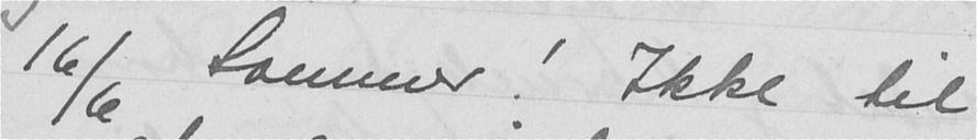16/6 Sommer! Ikke til
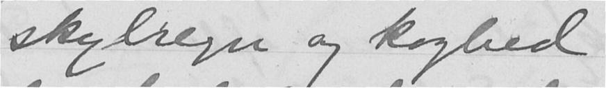skylregn og koghed
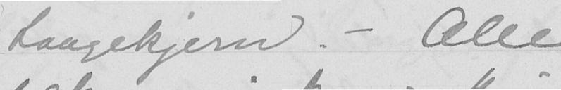Laagekjern. - Alle
